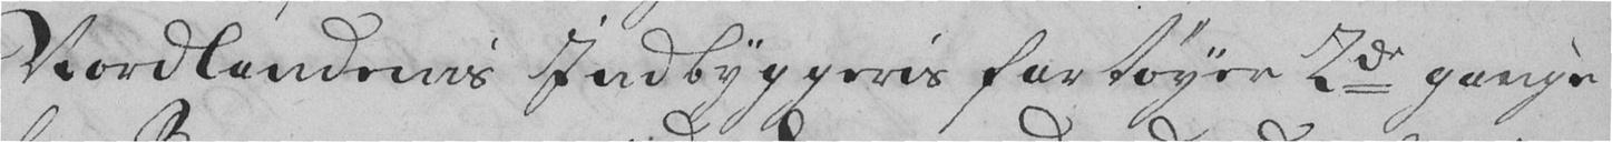Nordlandenis Indbyggeris fartøyer 2de gange
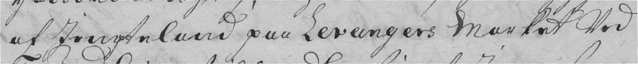af Iemteland paa Levangers Market Ved
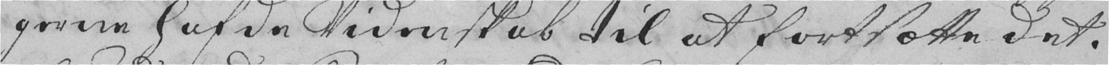gerne hafde Videnskab til at fortsætte det.
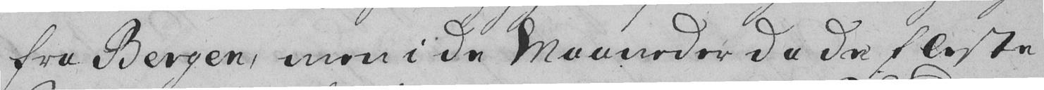fra Bergen, men i de Maaneder da de fleste
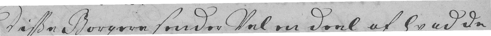Disse Borgere sender Vel en deel af hvad de
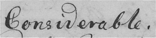Considerable.
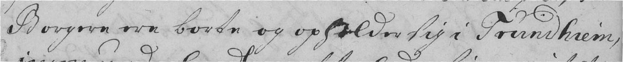Borgere ere borte og opholder sig i Trundhiem,
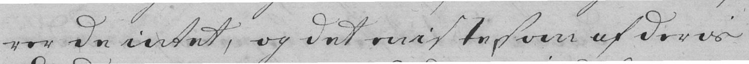rer de intet, og det eniste som af deris
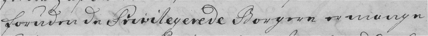Foruden de Privilegerede Borgere og mange
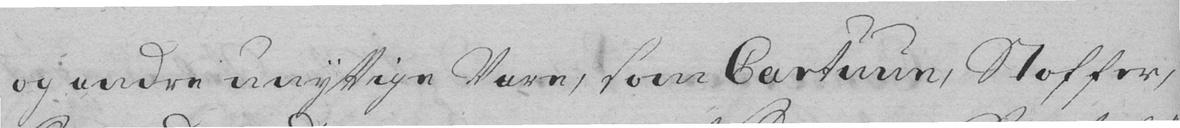og andre unyttige Vare, som Cartuun, Stoffer,
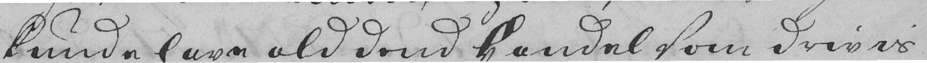kunde have ald dend Handel som drivis
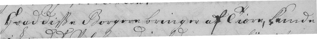Hvad disse Borgere bringer af Tiære, kunde
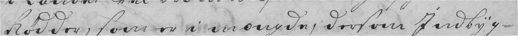Rødder, som er i mængde, dersom Jndbyg-
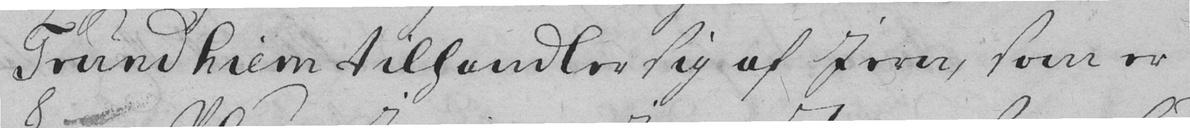Trundhiem tilhandler sig af Iern, som er
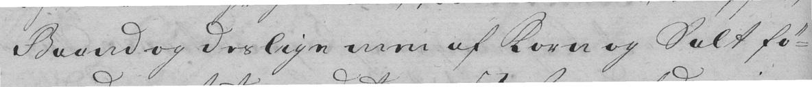Baand og deslige men af Korn og Salt fø-
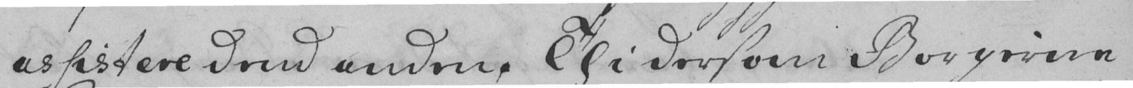assistere dend anden. Thi dersom Borgerne
